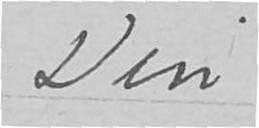Din
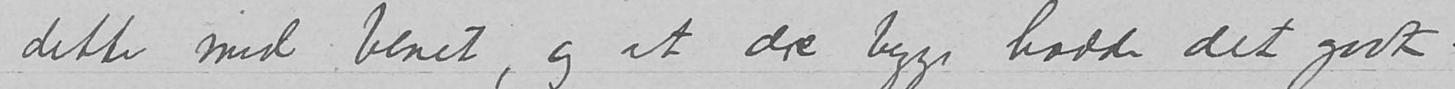dette med benet, og at dere begge hadde det godt.
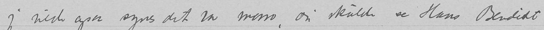jeg vilde ogsaa synes det var morro, du skulde se Hans Benedikt
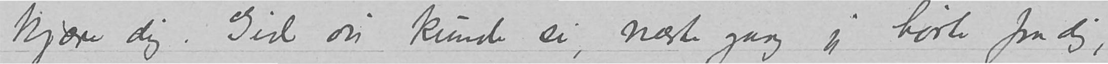kjære dig. Gid vi kunde si, næste gang jeg hørte fra dig,
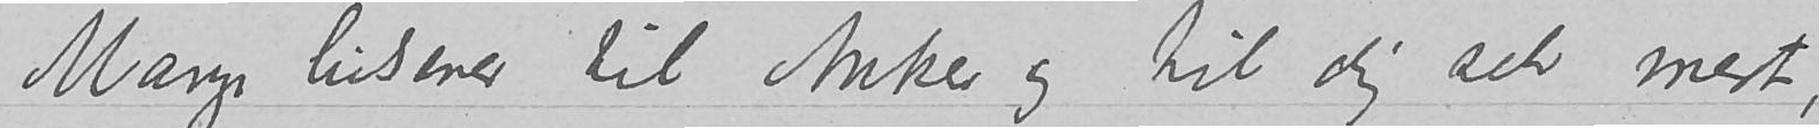Mange hilsener til Anker og til dig selv mest,
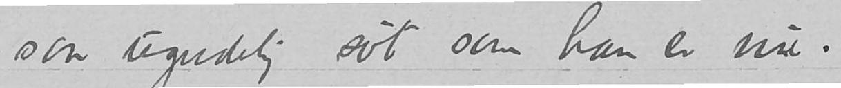saa ugudelig søt som han er nu.
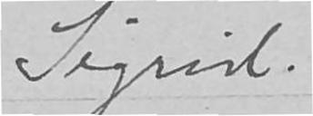Sigrid
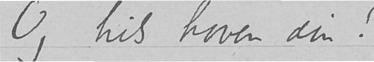Og hils haven din!
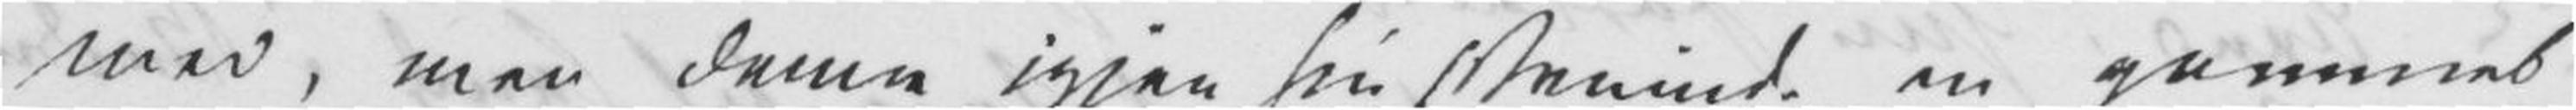med, men denne igjen sin Veninde, en gammel
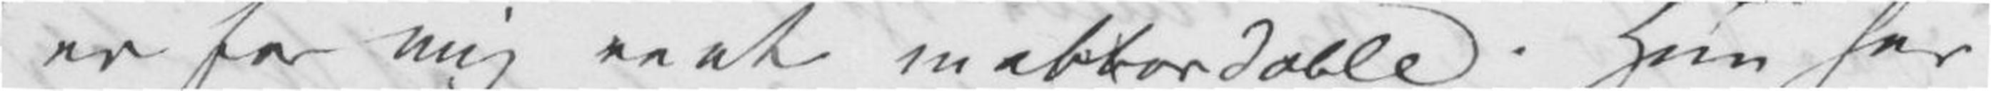er for mig rent inabbordable. Hun har
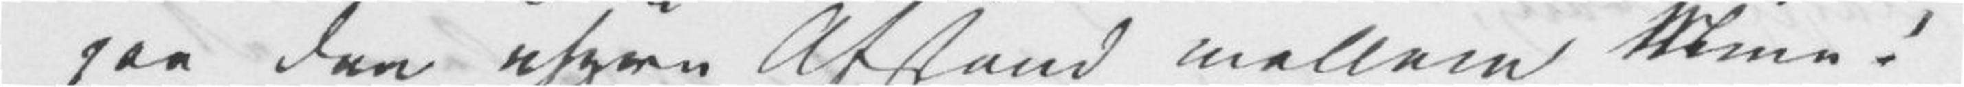paa den uhyre Afstand mellem Mine!
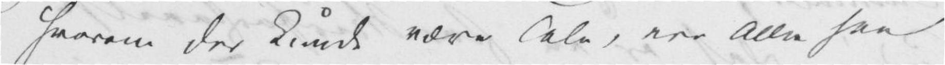hvorom der kunde være Tale, ere Alle saa
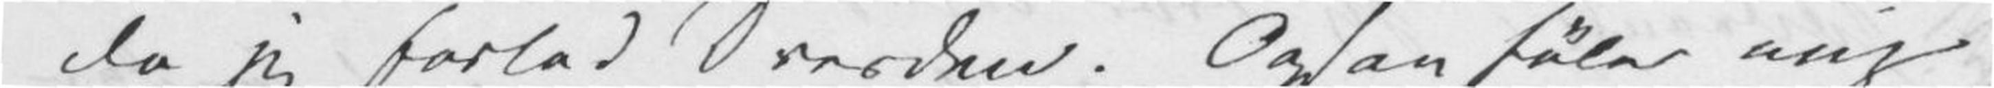da jeg forlod Dresden. Og saa føler [jeg] mig
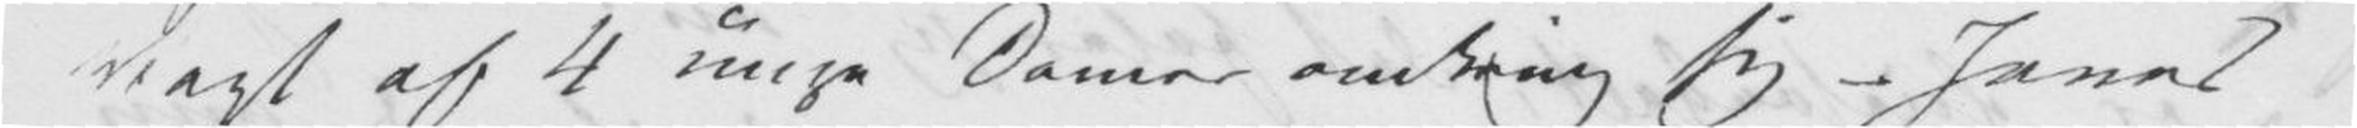Vagt af 4 unge Damer omkring sig – Jonas
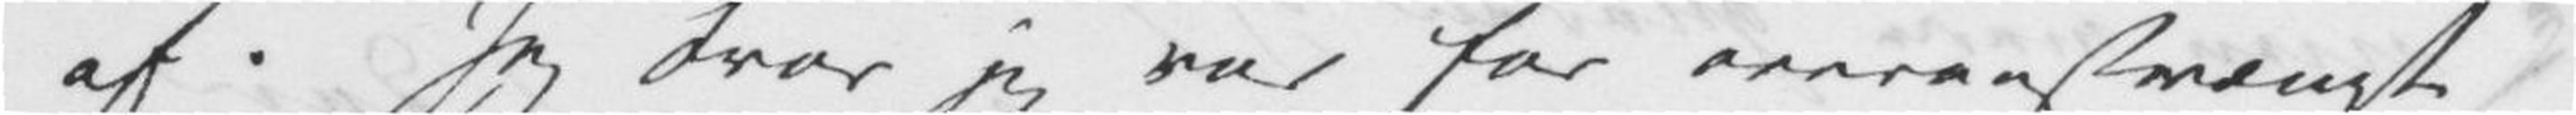af. Jeg tror jeg var for overanstrængt
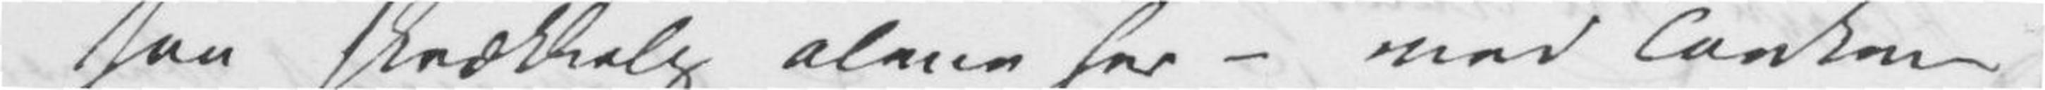saa skrækkelig alene her – med Tanken
